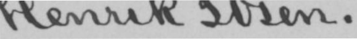Henrik Ibsen.
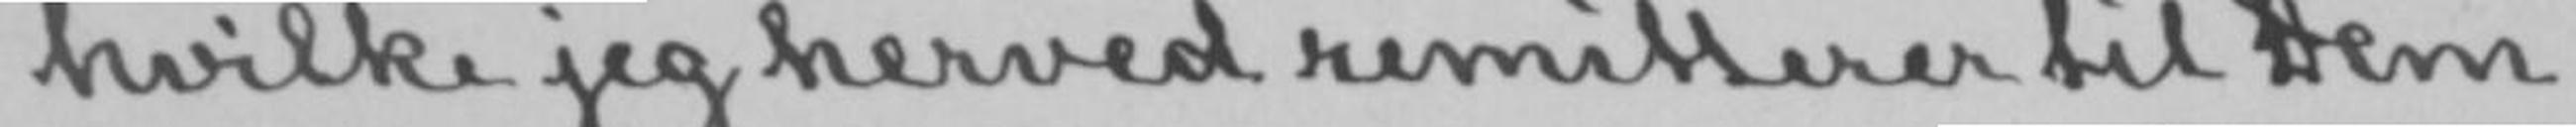hvilke jeg herved remitterer til Dem
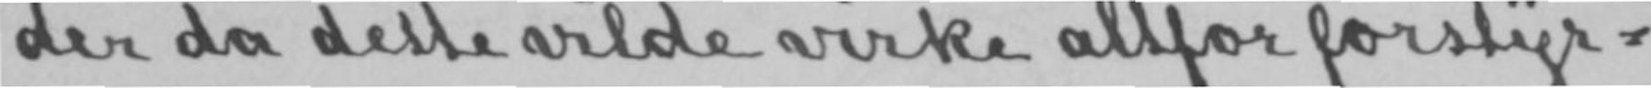der da dette vilde virke altfor forstyr-
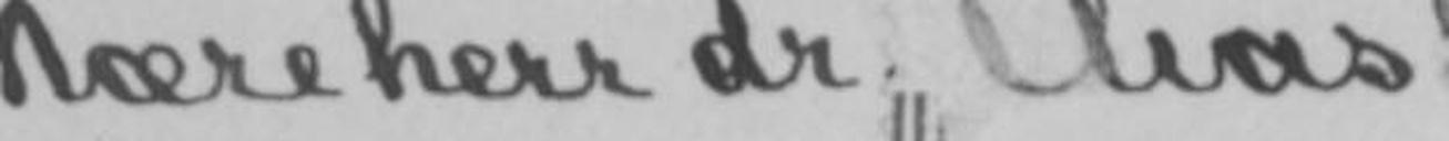Kære herr dr. Elias!
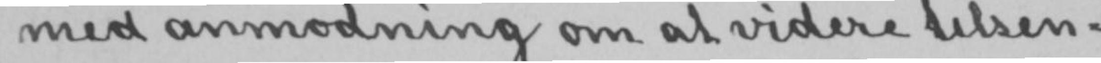med anmodning om at videre tilsen-
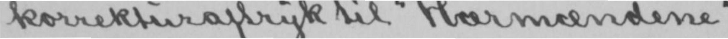korrekturaftryk til «Hærmændene»,
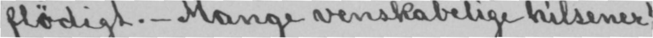flødigt.- Mange venskabelige hilsener!
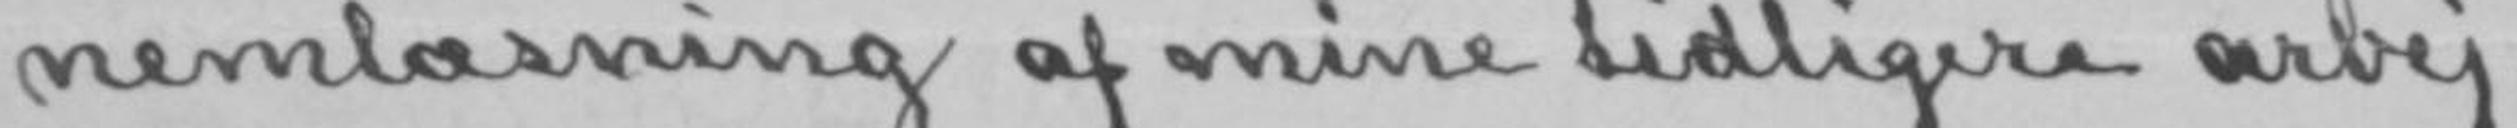nemlæsning af mine tidligere arbej-
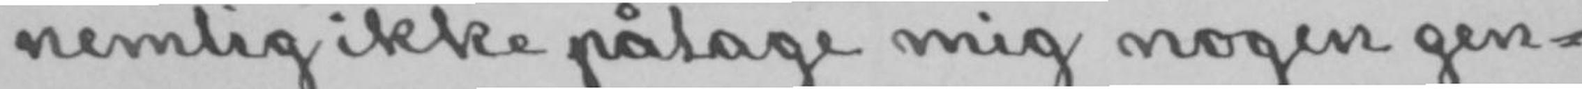nemlig ikke påtage mig nogen gen-
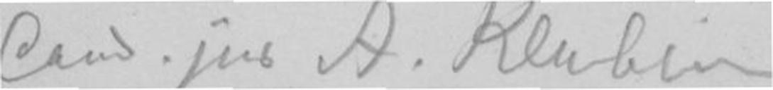Cand. jur A. Kluben
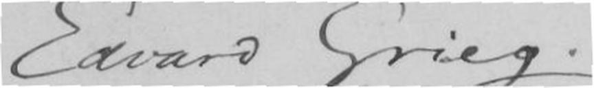Edvard Grieg.
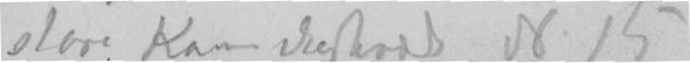Adr. Kandustrass N 15
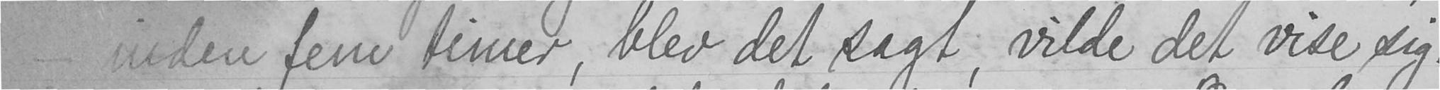- inden fem timer, blev det sagt, vilde det vise sig.
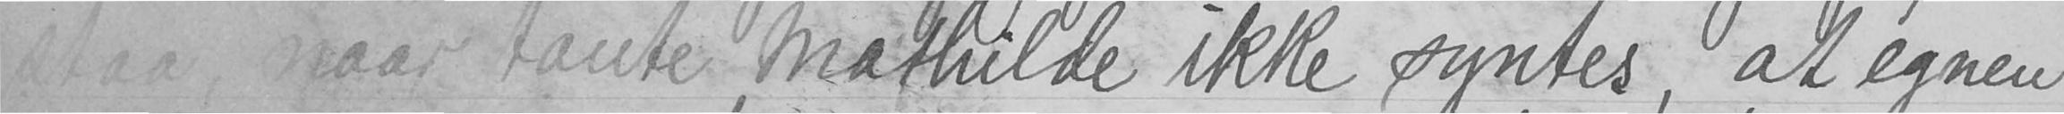staa, naar tante Mathilde ikke syntes, at egnen
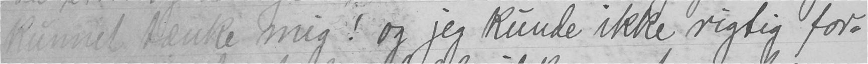kunnet tenke mig! og jeg kunde ikke rigtig for-
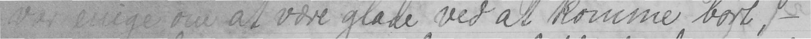var enige om at være glade ved at komme bort, -
